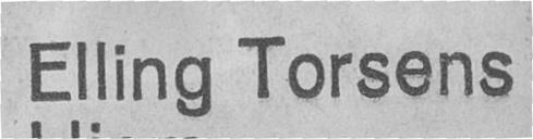Elling Torsens
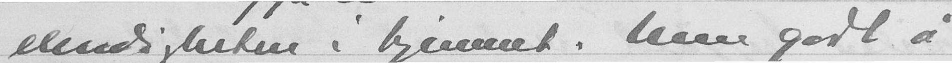elendigheten i hjemmet. Men godt å
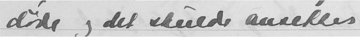døde og det skulde ansettes
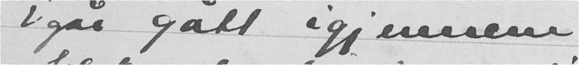igår gått igjennom
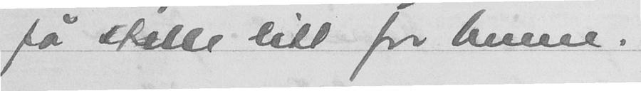få stelle litt for henne.
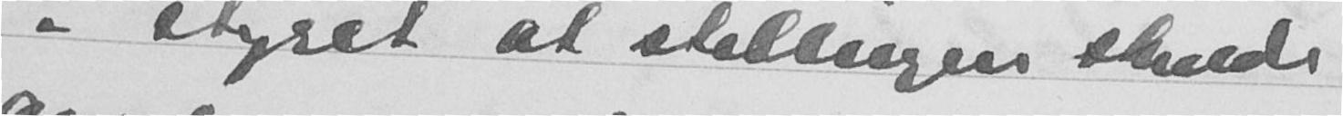i styret at stillingen skulde
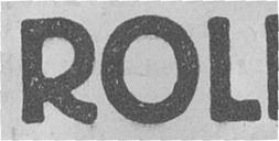ROLL
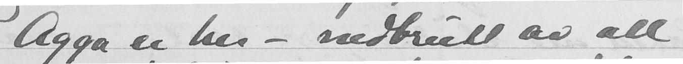Agga er her - nedbrutt av all
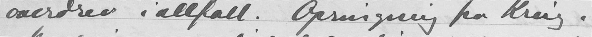overdrev iallfall. Opringning fra Krog.
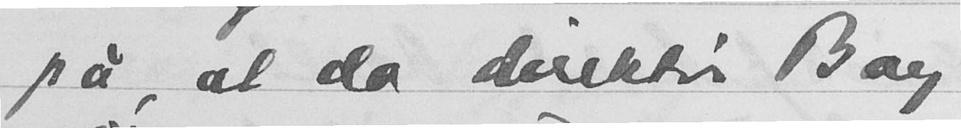på, at da direktør Bay
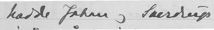hadde Johan og Sverdrups
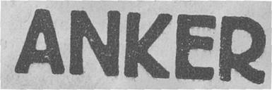ANKER
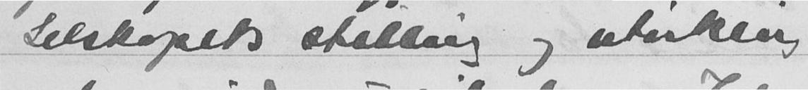Selskapets stilling og utvikling
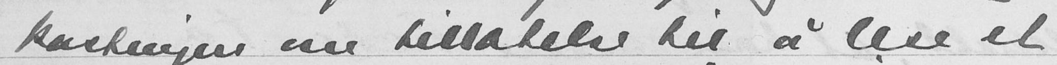kastingen om tillatelse til å lese et
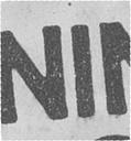NINI
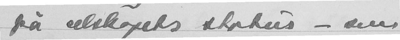på selskapets status - som
